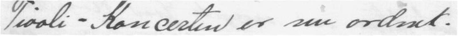Tivoli-Koncerten er nu ordnet.
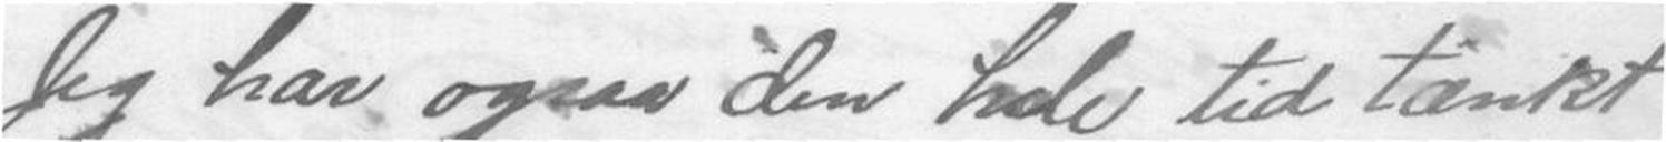Jeg har ogsaa den hele tid tænkt
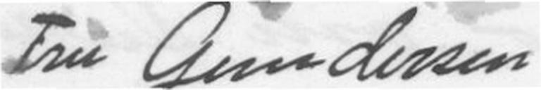Fru Gundersen
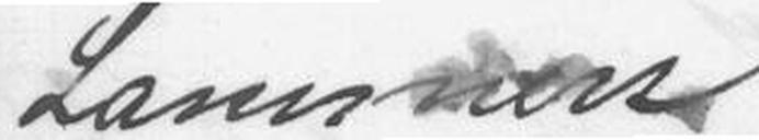Lammers
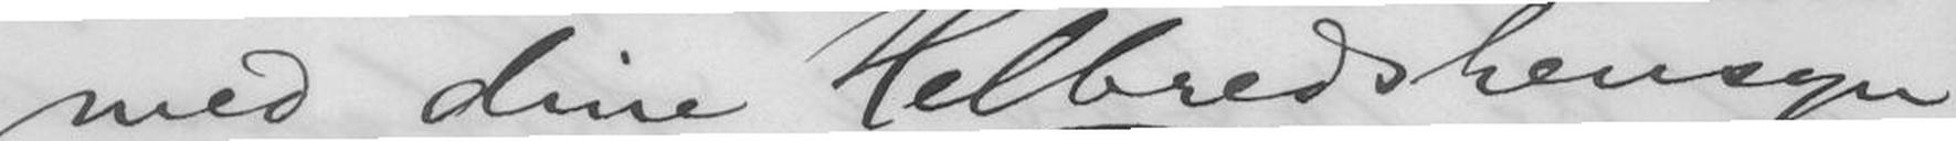med dine Helbredshensyn
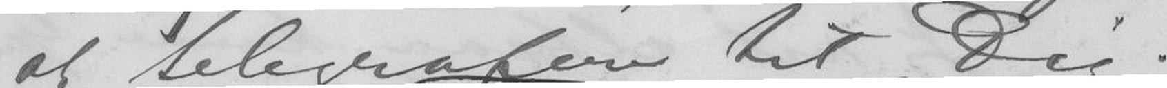at telegrafere til Dig.
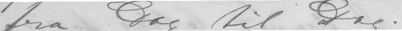fra Dag til Dag.
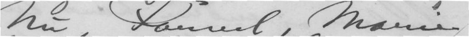Nu Farvel, Marie
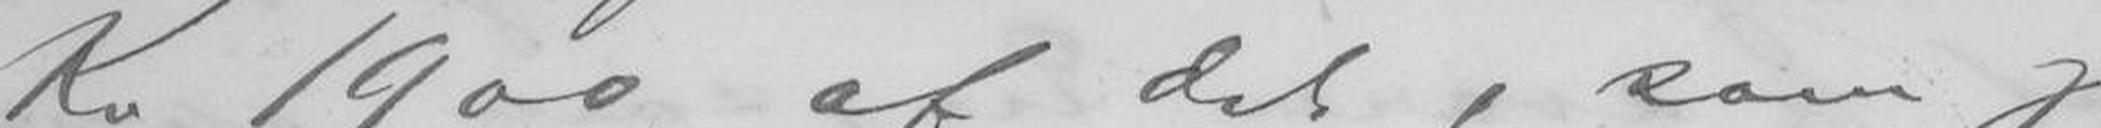Kr 1900 af det, som jeg
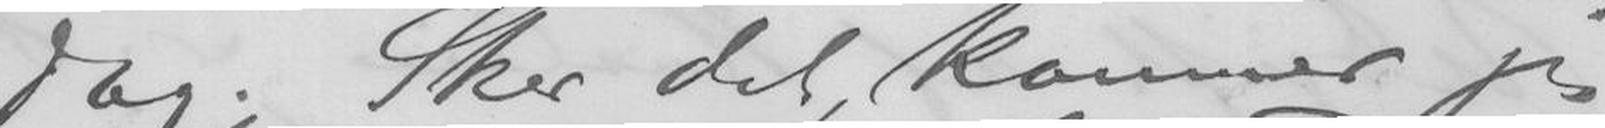dag. Sker det, kommer jeg
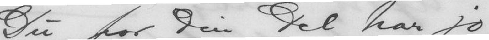Du for Din Del har jo
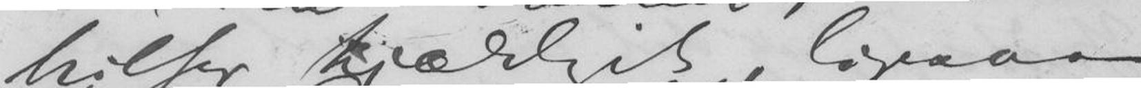hilser kjærligst, ligesaa
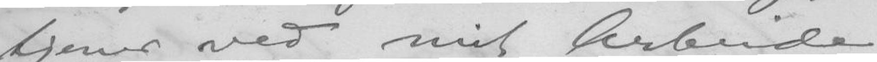tjener ved mit Arbeide
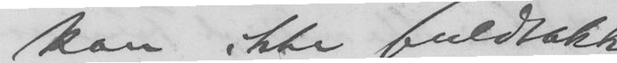kan ikke fuldtakke
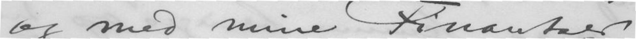og med mine Finantser.
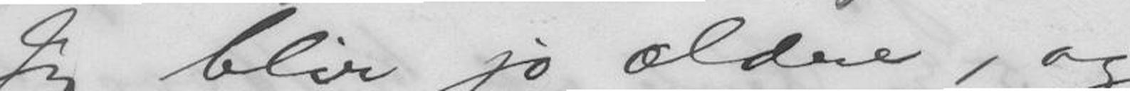Jeg blir jo ældre, og
